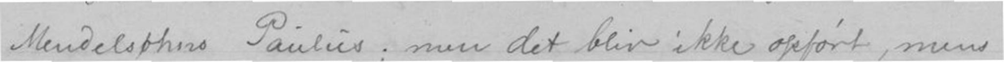Mendelsohns Paulus; men det blir ikke opført, mens
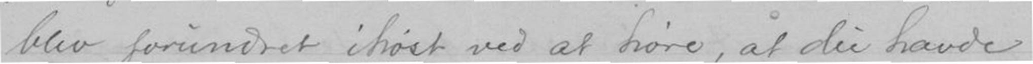blev forundret ihøst ved at høre, at du havde
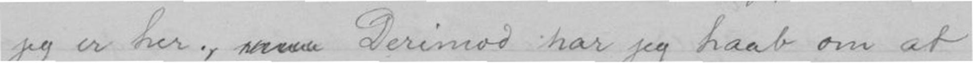jeg er her. Derimod har jeg haab om at
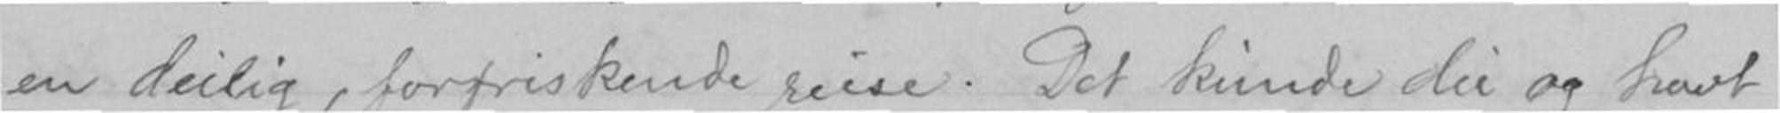en deilig, forfriskende reise. Det kunde du og havt
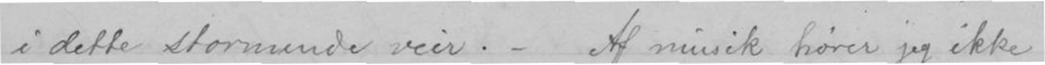i dette stormende veir. - Af musik hører jeg ikke
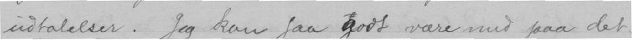udtalelser. Jeg kan saa godt være med paa det,
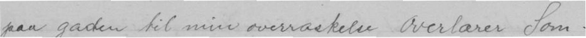paa gaden til min overraskelse Overlærer Som-
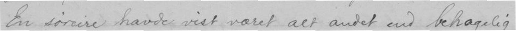En søreise havde vist været alt andet end behagelig
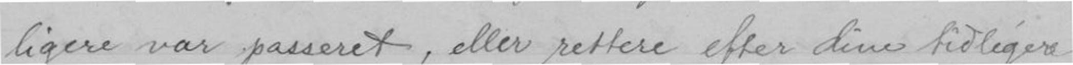ligere var passeret, eller rettere efter dine tidligere
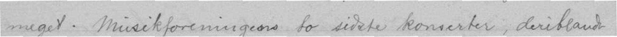meget. Musikforeningens to sidste konserter, deriblandt
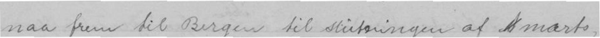naa frem til Bergen til slutningen af marts,
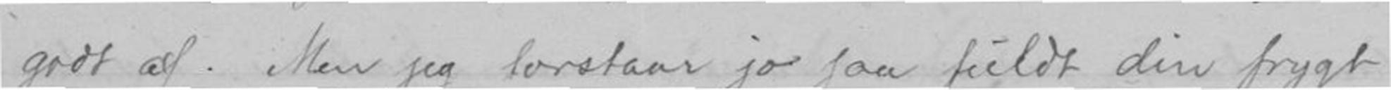godt af. Men jeg forstaar jo saa fuldt din frygt
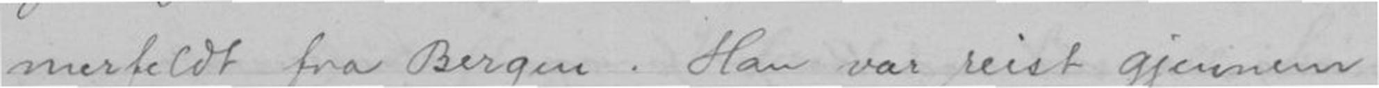merfeldt fra Bergen. Han var reist gjennem
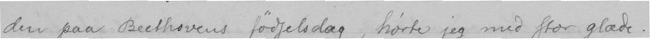den paa Beethovens fødselsdag, hørte jeg med stor glæde;
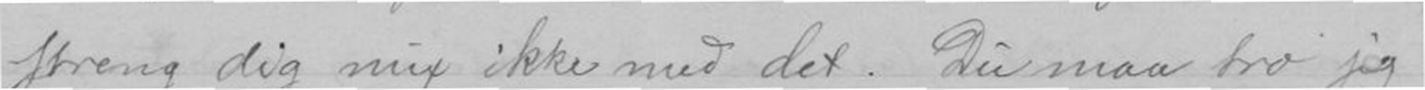streng dig nu ikke med det. Du maa tro jeg
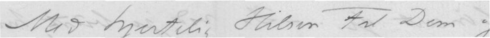Med hjertelig Hilsen til Dem og
